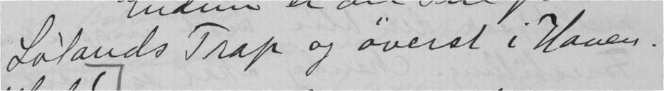Lølands Trap og øverst i Haven.
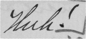Huh!
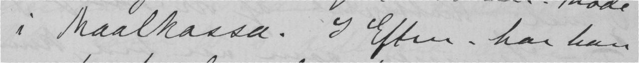i Maalkassa. I Eftm. har han
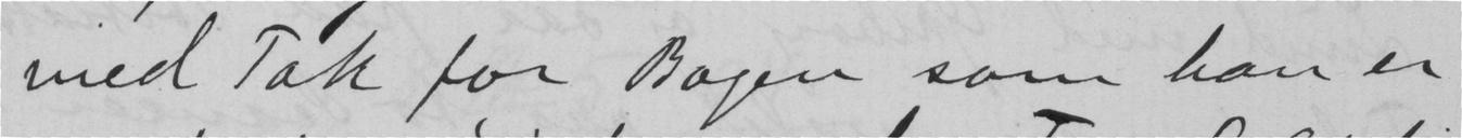med Tak for Bogen som han er
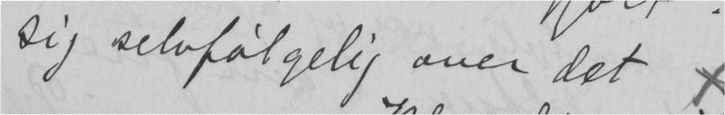sig selvfølgelig over det x
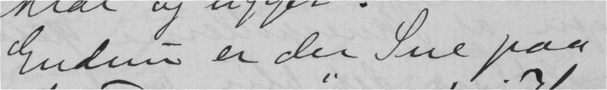Endnu er der Sne paa
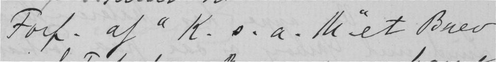Forf. af "K. s. a. M" et Brev
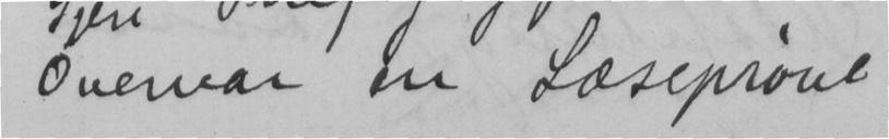Overvar en Læseprøve
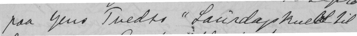paa Jens Tvedts "Laurdagskveld til
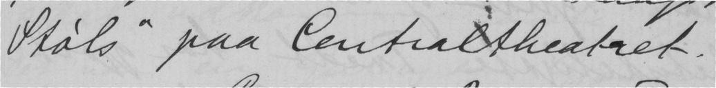Støls" paa Centraltheatret.
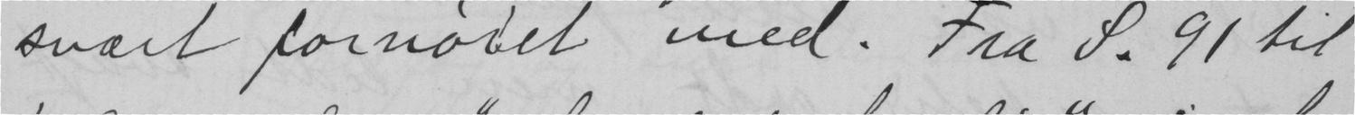svært fornøiet med. Fra S. 91 til
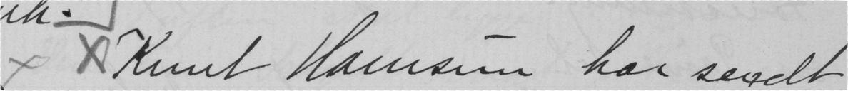x Knut Hamsun har sendt
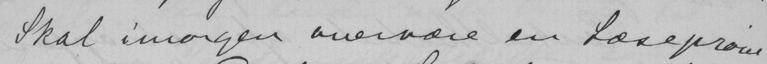Skal imorgen overvære en Læseprøve
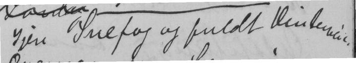Igjen Snefog og fuldt Vinterveir.
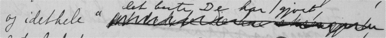og idethele "
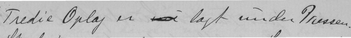Tredie Oplag er  lagt under Pressen.
